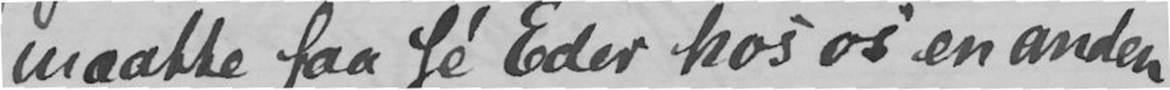maatte faa se Eder hos os en anden
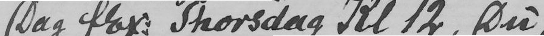Dag fex: Thorsdag Kl 12, Du,
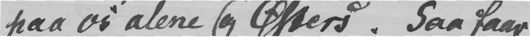paa os alene og Østers. Saa faar
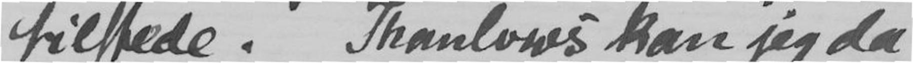tilstede. Thaulows kan jeg da
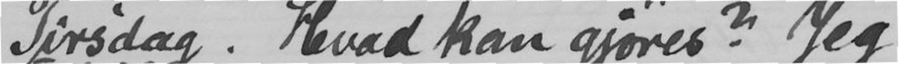Tirsdag. Hvad kan gjøres? Jeg
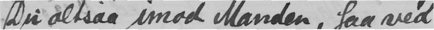Du altsaa imod Manden, saa ved
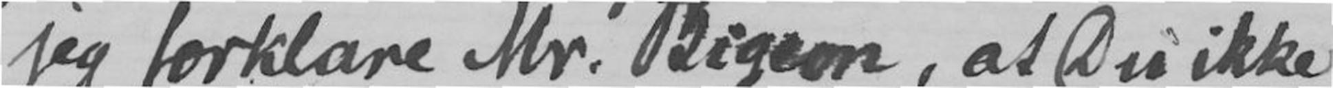jeg forklare Mr.Bigeon, at Du ikke
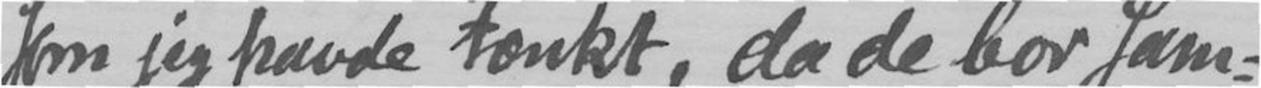som jeg havde tænkt, da de bor sam-
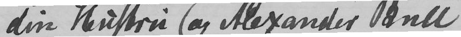din Hustru og Alexander Bull
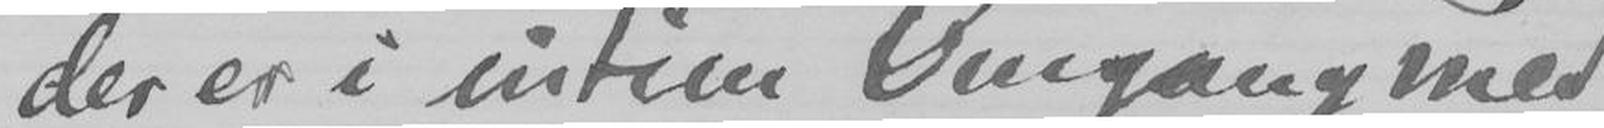der er i intim Omgang med
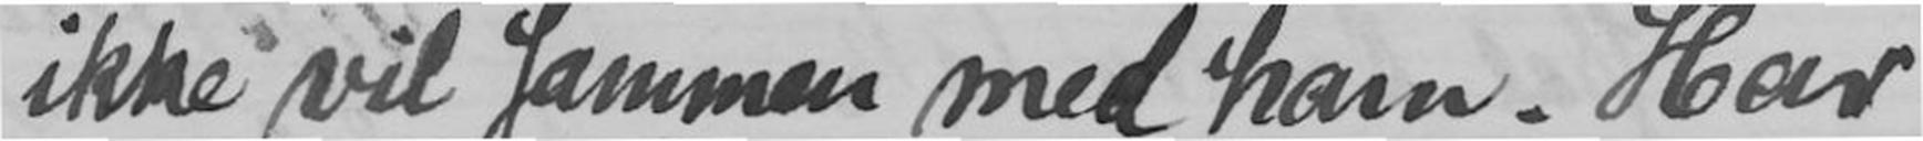ikke vil sammen med ham. Har
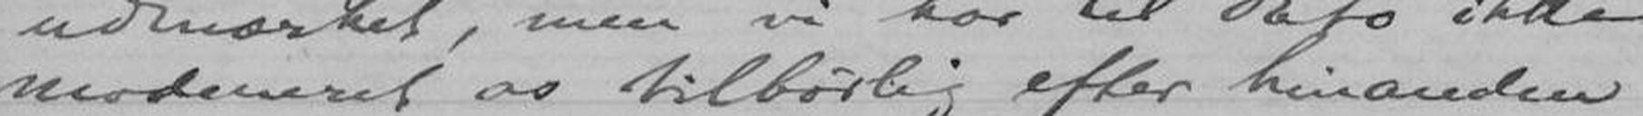modereret os tilbørlig efter hinanden,
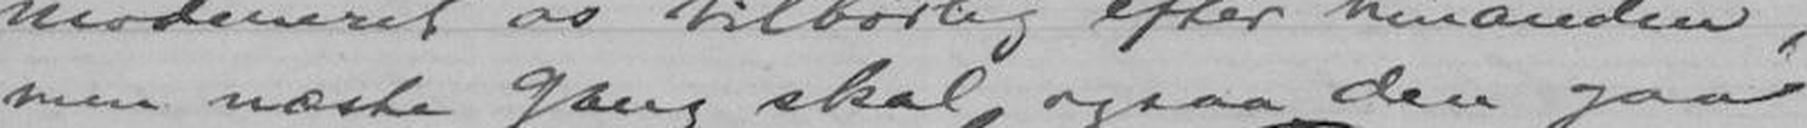men næste Gang skal ogsaa den gaa
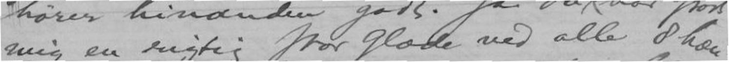mig en rigtig stor Glæde ved alle 8 Hæn-
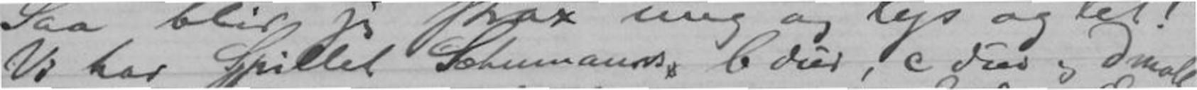Vi har spillet Schumanns b dur, c dur og dmoll,
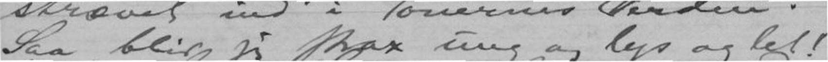Saa blir jeg strax ung og lys og let!
